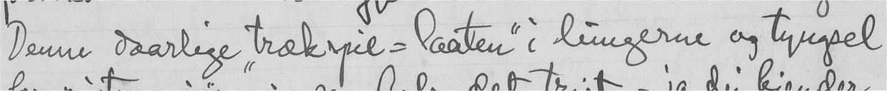Denne daarlige "trækspil-laaten" i lungerne og tyngsel
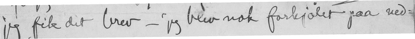jeg fik dit brev - jeg blev nok forkjølet paa ned-
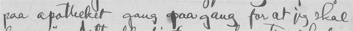paa apotheket gang paa gang for at jeg skal
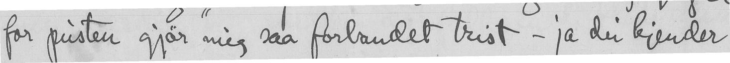for pusten gjør mig saa forbandet trist - ja du kjender
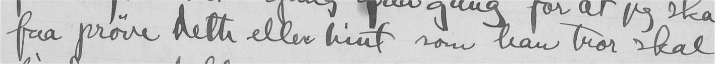faa prøve dette eller hint som han tror skal
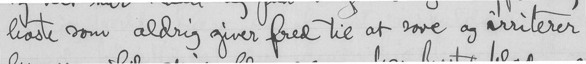hoste som aldrig giver fred til at sove og irriterer
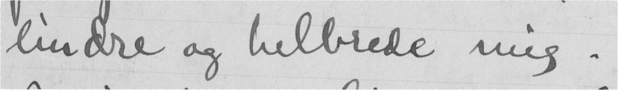lindre og helbrede mig.
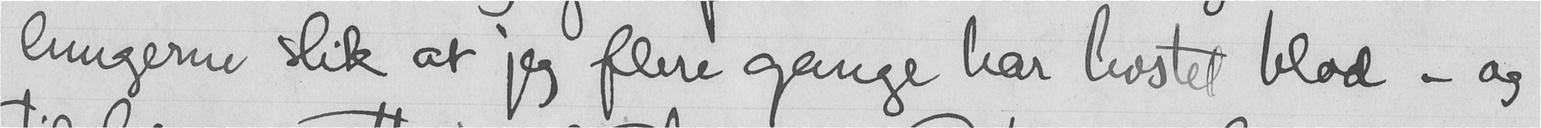lungerne slik at jeg flere gange har hostet blod - og
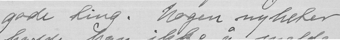gode ting. Nogen nyheter
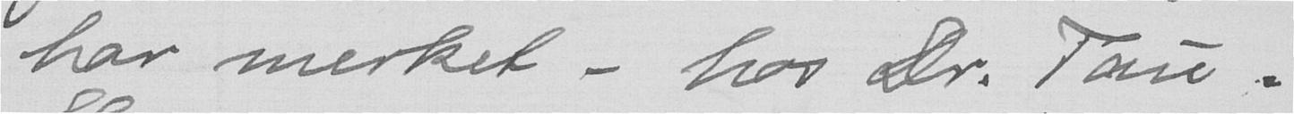har merket - hos dr. Tau.
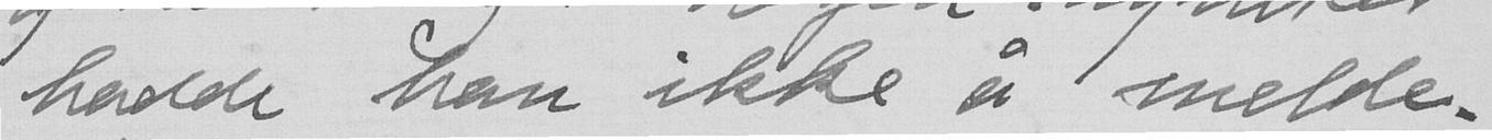hadde han ikke å melde.
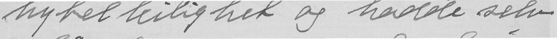hybelleilighet og hadde selv
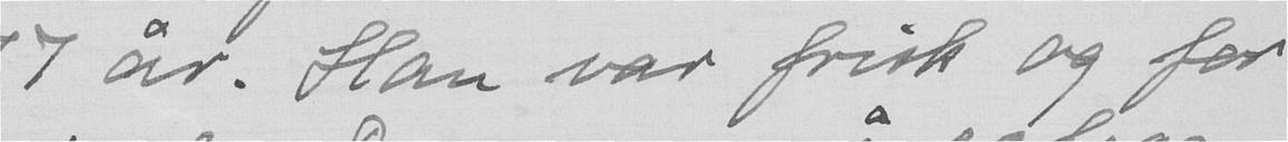77 år. Han var frisk og for
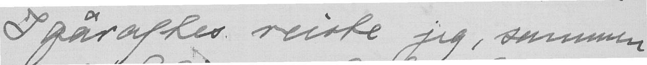I gåraftes reiste jeg, sammen
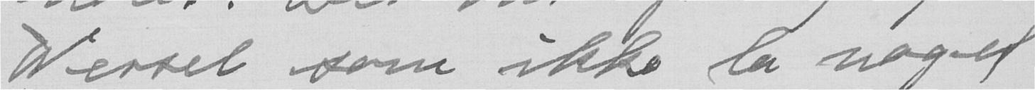Wessel som ikke la noget
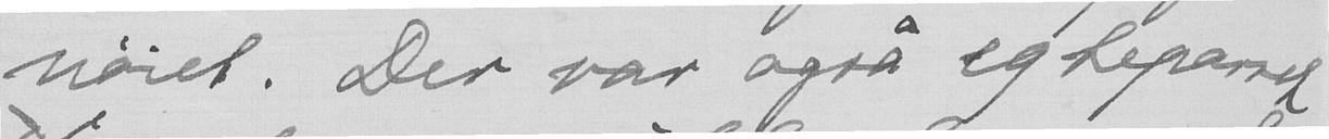nøiet. Der var også egteparret
